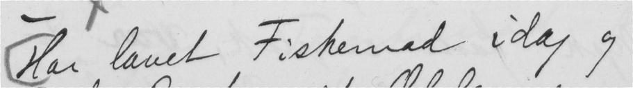Har lavet Fiskemad idag og
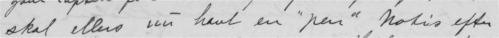skal ellers nu havt en "pen" Notis efter
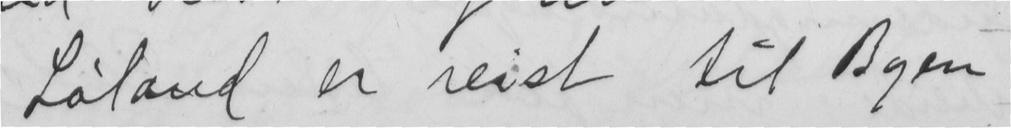Løland er reist til Byen
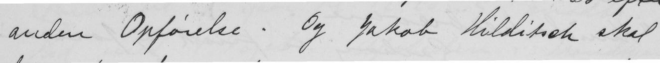anden Opførelse. Og Jakob Hilditsch skal
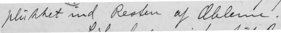plukket ind Resten af Æblerne.
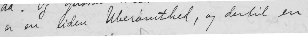er en liden Uberømthed, og dertil en
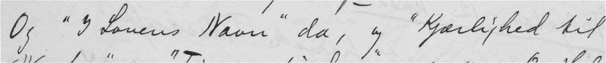Og "I Lovens Navn" da, og "Kjærlighed til
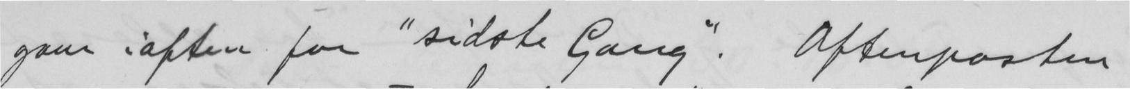gaar iaften for "sidste Gang". Aftenposten
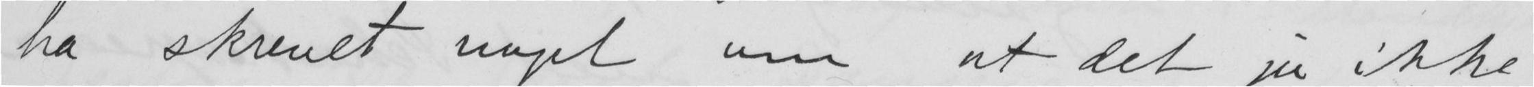ha skrevet noget um, at det jo ikke
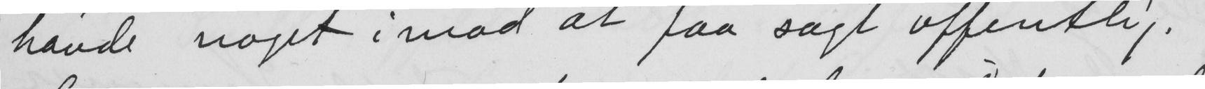havde noget imod at faa sagt offentlig.
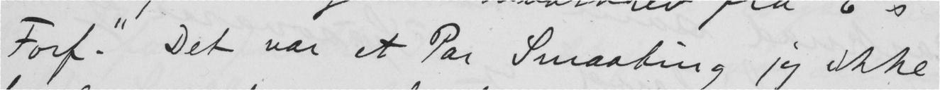Forf." Det var et Par Smaating jeg ikke
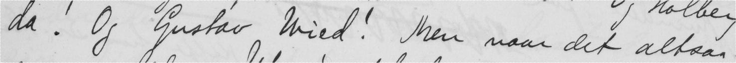da! Og Gustav Wied! Men naar det altsaa
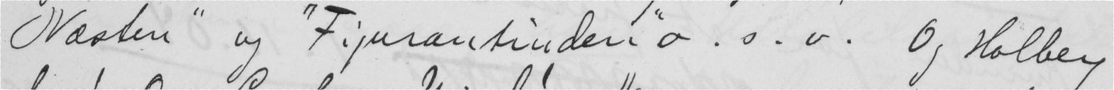Næsten" og "Fijurantinden" o. s. v. Og Holberg
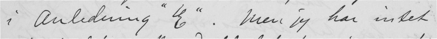i Anledning "E". Men jeg har intet
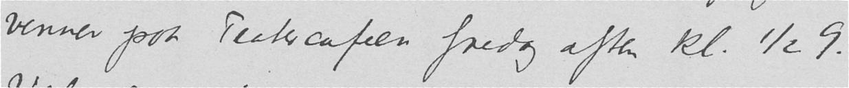venner paa Teatercafeen fredag aften kl. ½ 9.
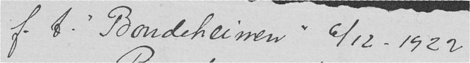f. t. "Bondeheimen" 6/12-1922
